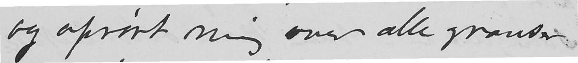og oprørt mig over alle grænser.
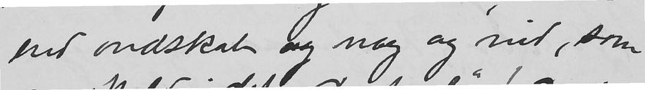end ondskab og nag og vid, som
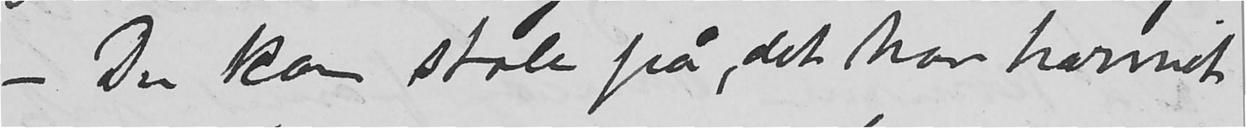- Du kan stole på, det han harmet
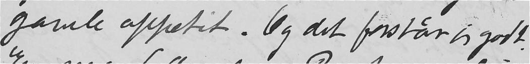gamle appetit. Og det forstår jeg godt.
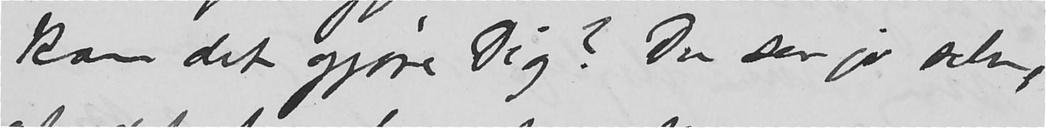kan det gjøre Dig? Du ser jo selv,
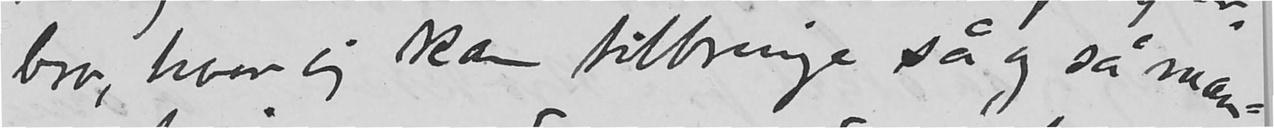bro, hvor jeg kan tilbringe så og så man-
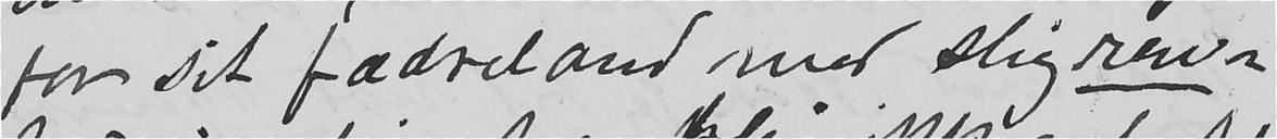for sit fædreland med slig ren-
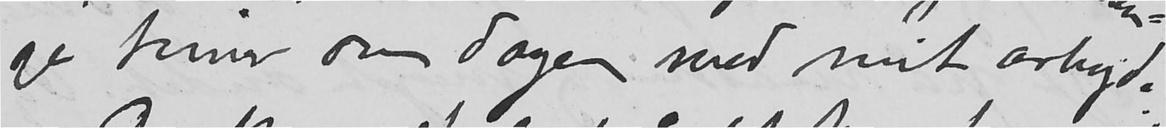ge timer om dagen med mit arbejd.-
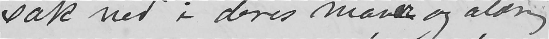sak ned i deres maver og aldrig
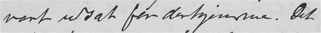vært udsat for derhjemme. Det
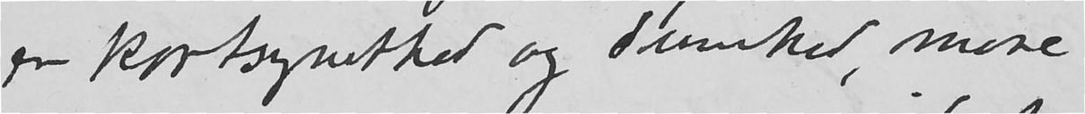er kortsynethed og dumhed, mere
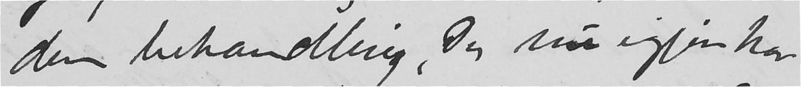den behandling, Du nu igjen har
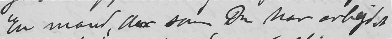En mand, der som Du har arbejdet
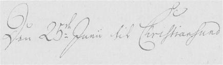Den 25te Junii til Christiansund
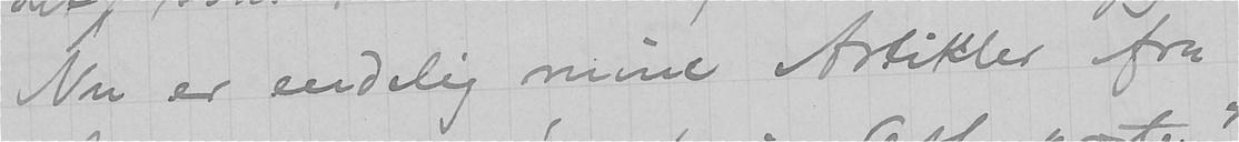Nu er endelig mine Artikler fra
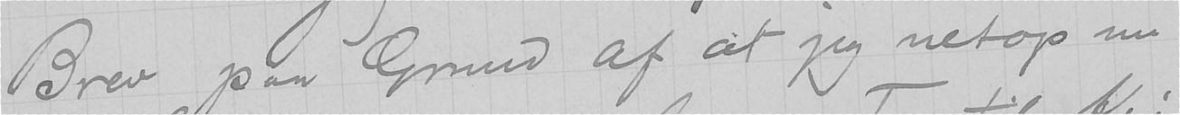Brev paa Grund af at jeg netop nu
